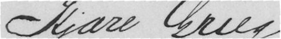Kjære Grieg
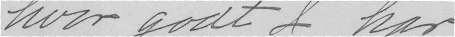hvor godt I har
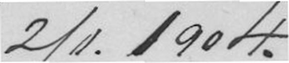2/1. 1904.
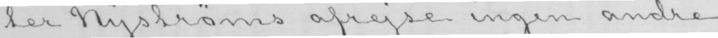ter Nyströms afrejse ingen andre
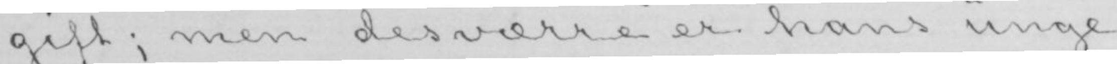gift; men desværre er hans unge
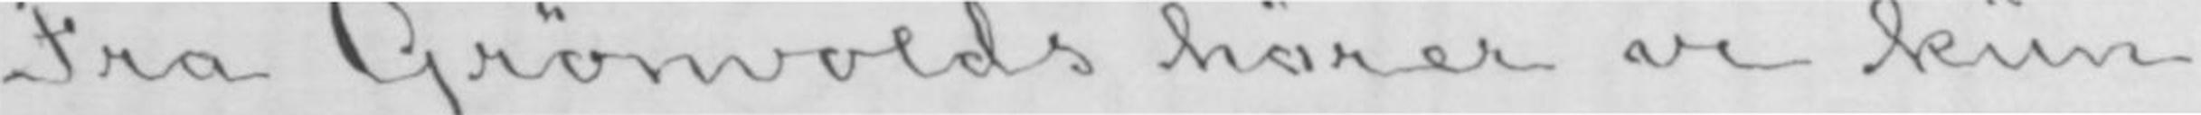Fra Grønvolds hører vi kun
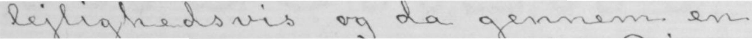leilighedsvis og da gennem en
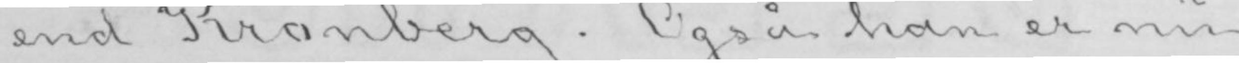end Kronberg. Også han er nu
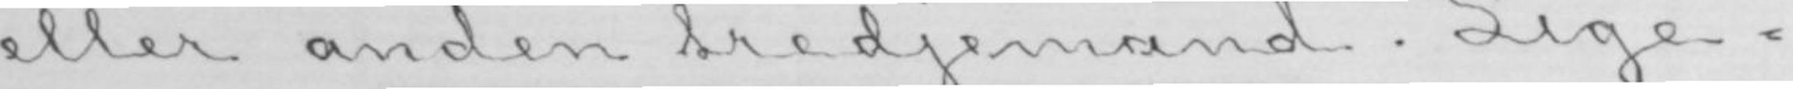eller anden tredjemand. Lige-
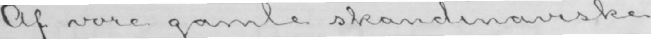Af vore gamle skandinaviske
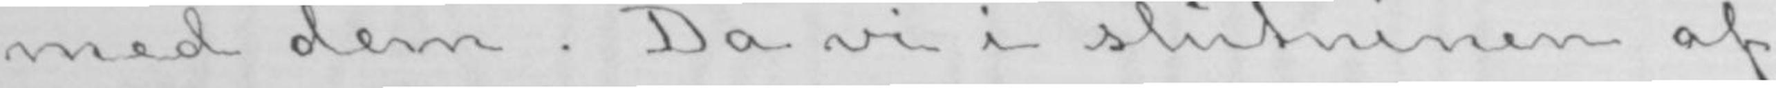med dem. Da vi i slutningen af
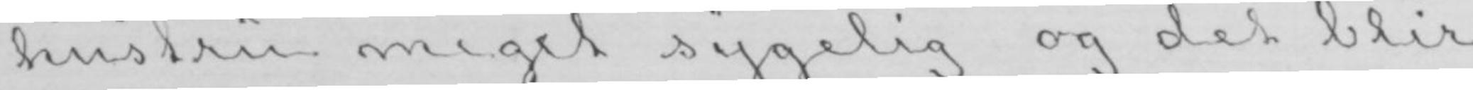hustru meget sygelig og det blir
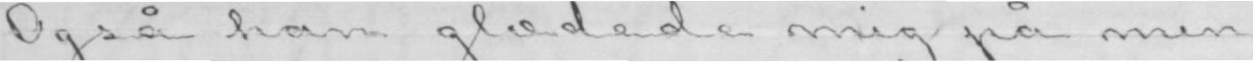Også han glædede mig på min
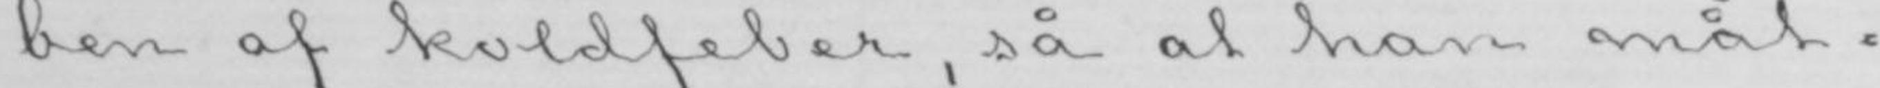ben af koldfeber, så at han måt-
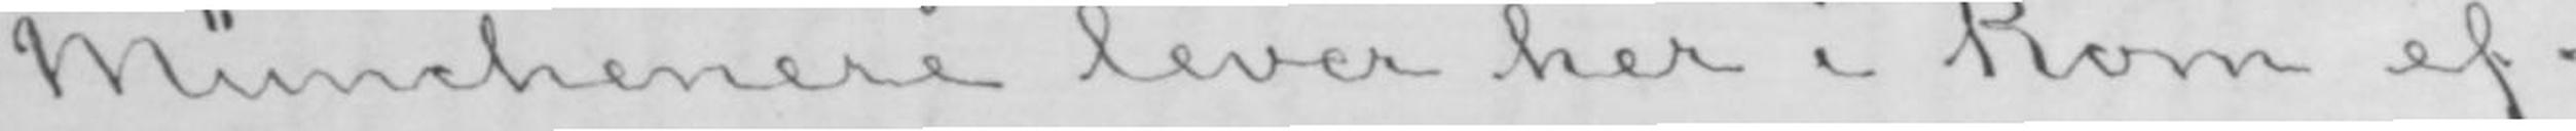Münchenere lever her i Rom ef-
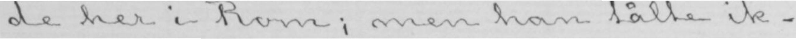de her i Rom; men han tålte ik-
